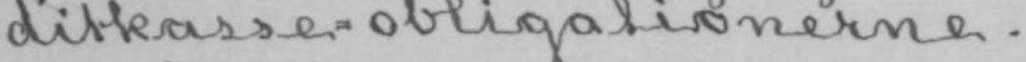ditkasse-obligationerne.
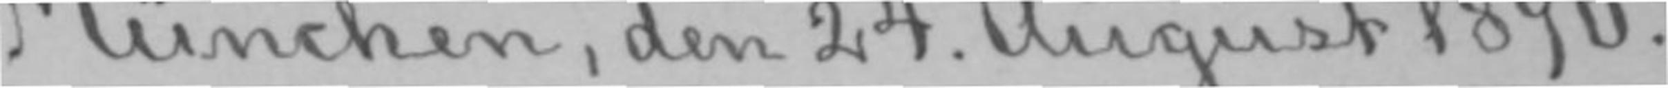München, den 24. August 1890.
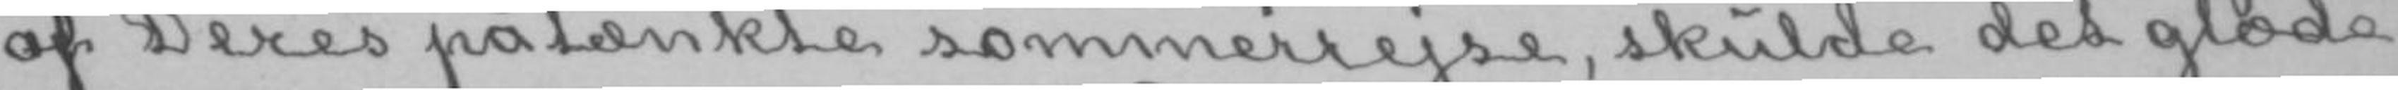af Deres påtænkte sommerrejse, skulde det glæde
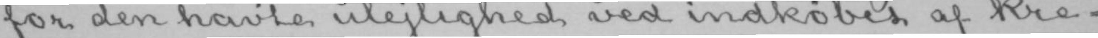for den havte ulejlighed ved indkøbet af kre-
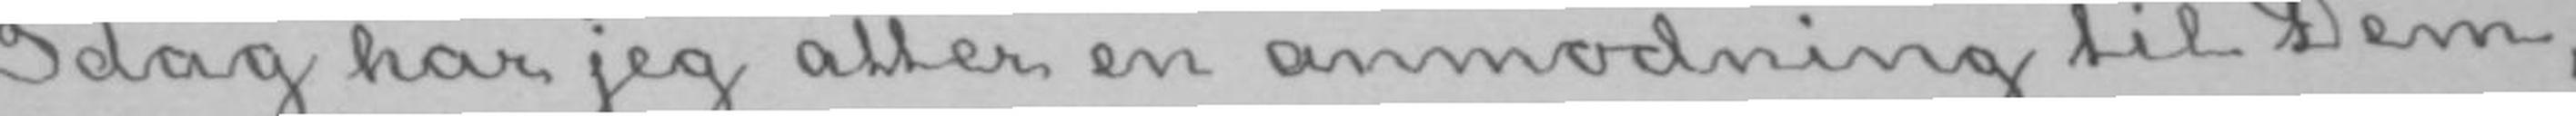Idag har jeg atter en anmodning til Dem,
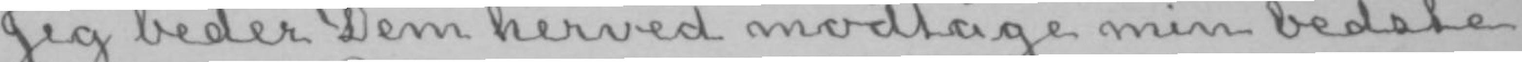Jeg beder Dem herved modtage min bedste
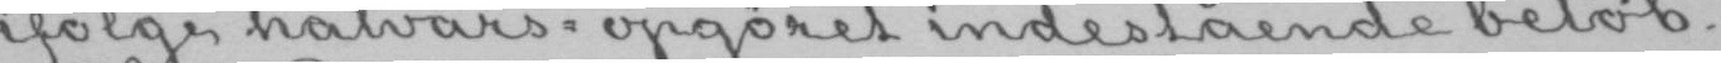ifølge halvårs-opgøret indestående beløb.
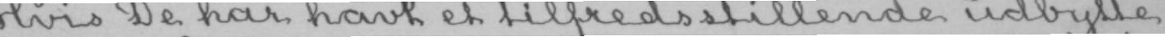Hvis De har havt et tilfredsstillende udbytte
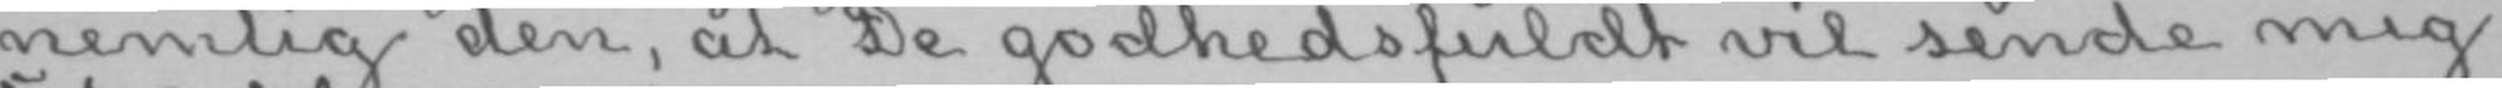nemlig den, at De godhedsfuldt vil sende mig
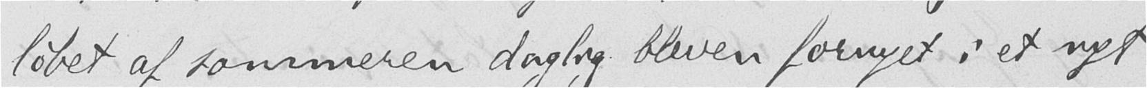løbet af sommeren daglig bleven fornyet i et nyt
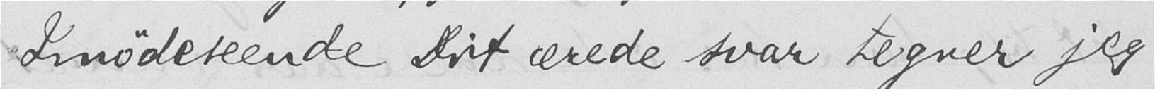Imødeseende Dit ærede svar tegner jeg
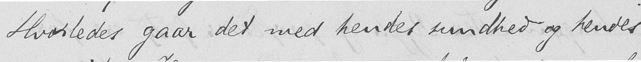Hvorledes gaar det med hendes sundhed og hendes
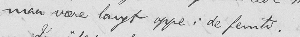maa være langt oppe i de femti.
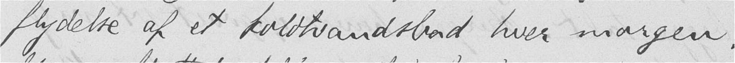flydelse af et koldtvandsbad hver morgen.
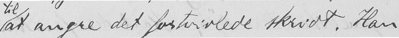at angre det fortvivlede skridt. Han
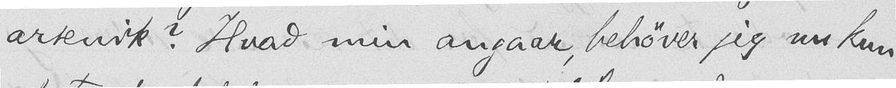arsenik? Hvad min angaar, behøver jeg nu kun
